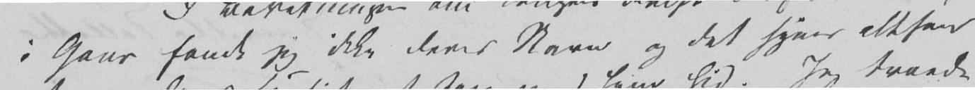i Gaar fandt jeg ikke Deres navn og det synes altsaa
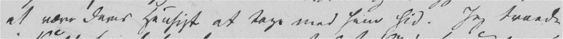at være Deres Hensigt at tage med ham hid. Jeg troede
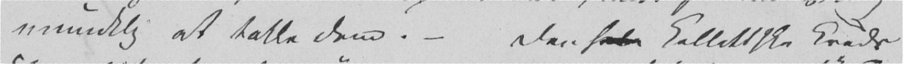mundtlig at takke Dem. – Den hele Collettske Kreds
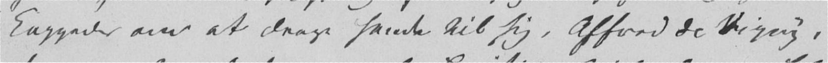kappedes om at drage hende til sig, Alfredde Vigny,
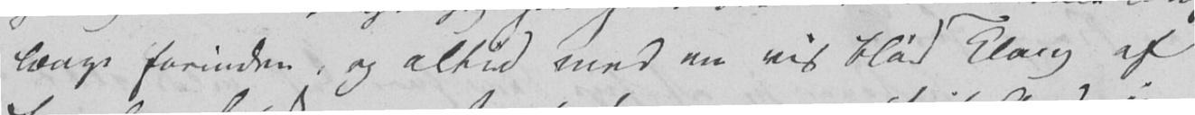længe forinden, og altid med en vis blød Klang af
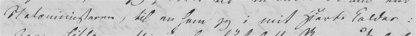Statsministeren, til en som jeg i mit Hjerte kalder:
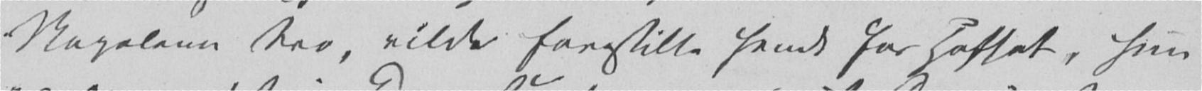Napoleon tro, vilde forestille hende for Hoffet, hun
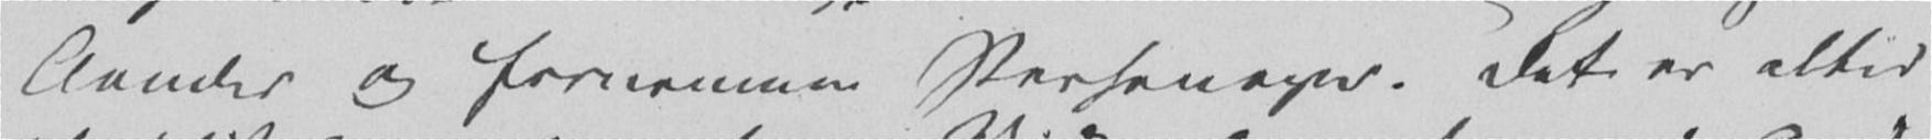Aander og fornemme Personager. Det er altid
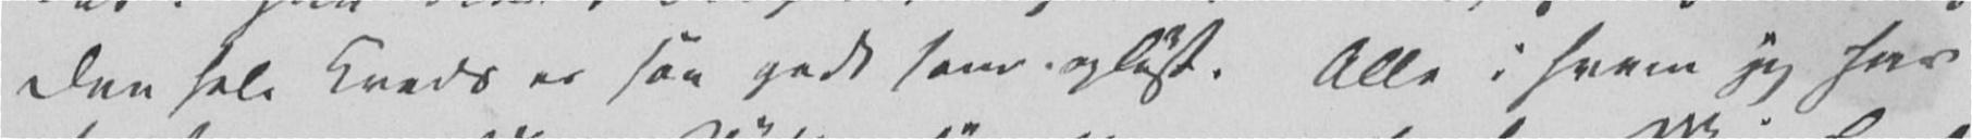Den hele Kreds er saa godt som opløst. Alle i hvem jeg har
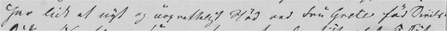har lidt et nyt og uopretteligt Stød ved Fru Grete fød Diriks'
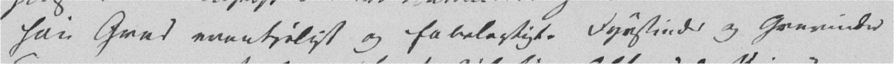høi Grad eventyrligt og fabelagtigt. Fyrstinder og Grevinder
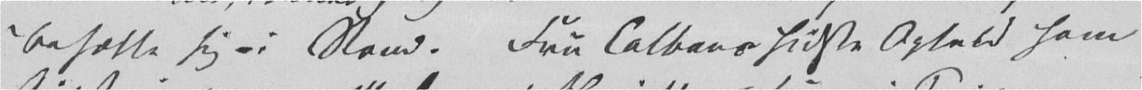«bosætte sig» i Rom. Fru Colbans sidste Ophold som
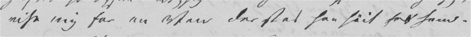vise mig for en Ven der stod saa høit for ham.
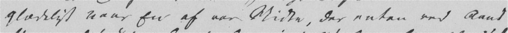glædeligt naar En af vor Midte, der enten ved Aand
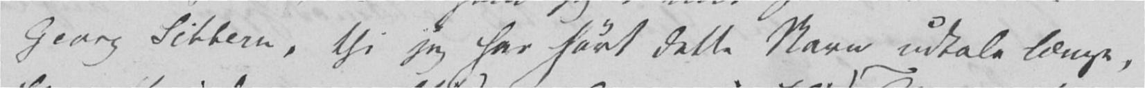Georg Sibbern, thi jeg har hørt dette Navn udtale længe,
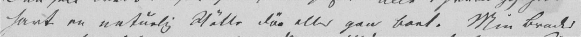havt en naturlig Støtte døe eller gaa bort. Min Broder
